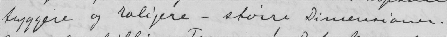tryggere og roligere — større Dimensioner.
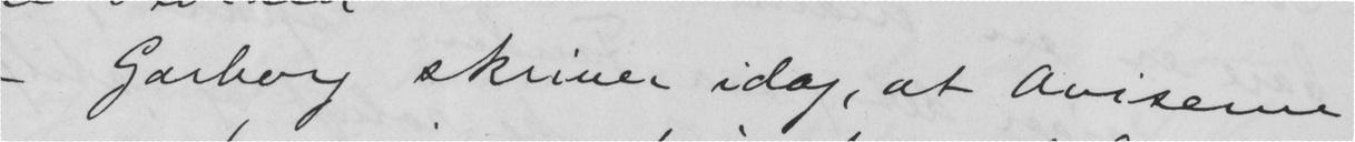- Garborg skriver idag, at Aviserne
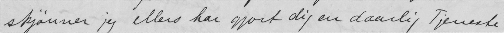skjønner jeg ellers har gjort dig en daarlig Tjeneste
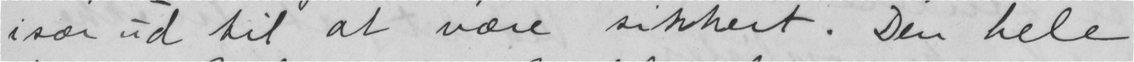især ud til at være sikkert. Den hele
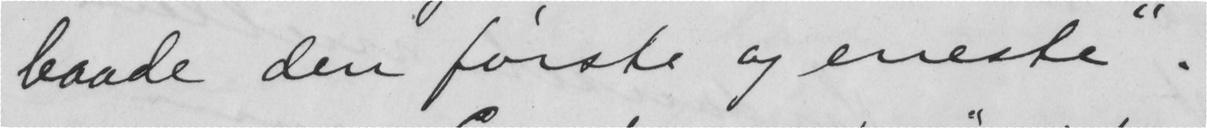baade den første og eneste".
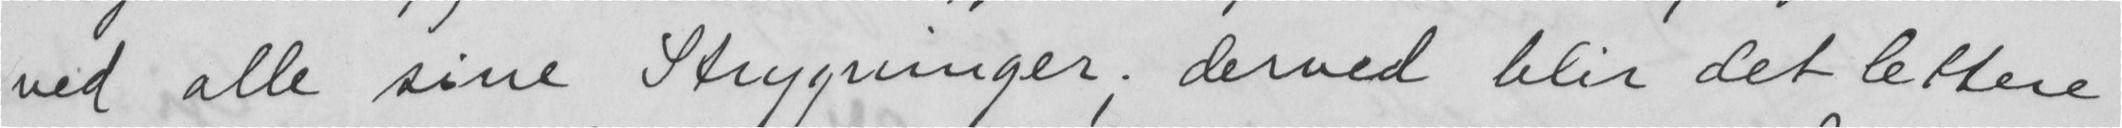ved alle sine Strygninger; derved blir det lettere
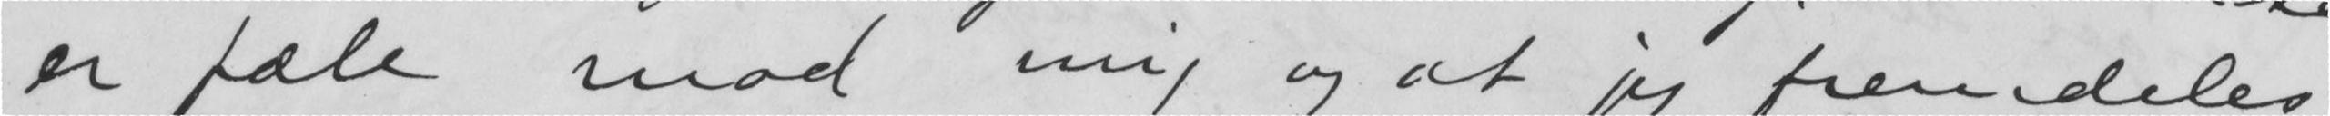er fæle mod mig og at jeg fremdeles
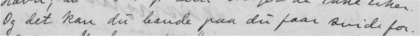Og det kan du bande paa du faar svide for.
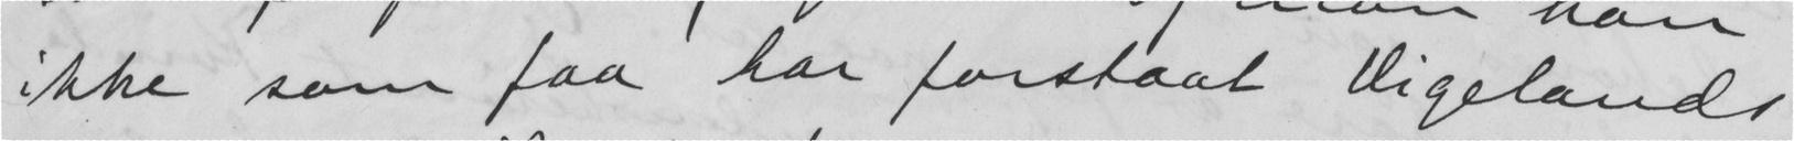ikke som faa har forstaat Vigelands
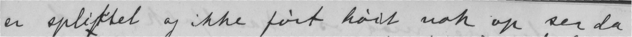er splittet og ikke ført høit nok op ser da
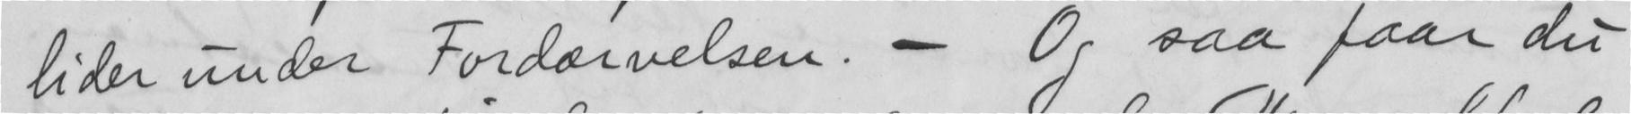lider under Fordærvelsen. — Og saa faar du
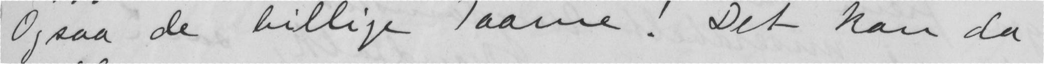Ogsaa de billige Taarne! Det kan da
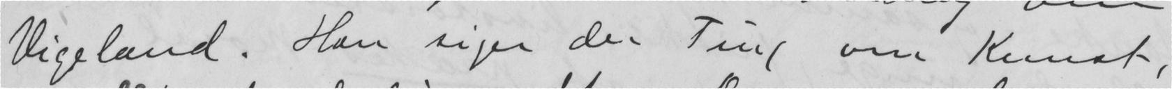Vigeland. Han siger der Ting om Kunst,
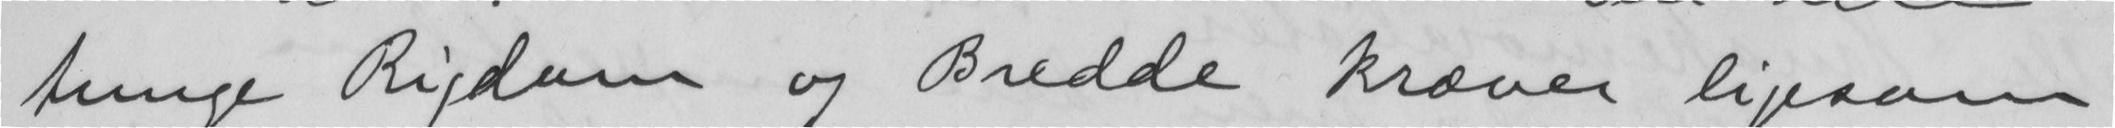tunge Rigdom og Bredde kræver ligesom
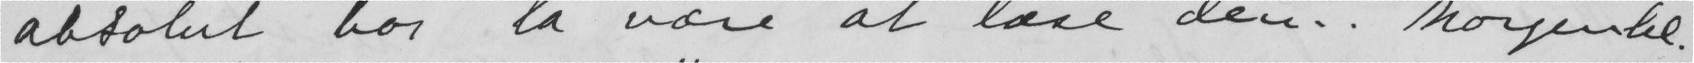absolut bør la være at læse dem. Morgentels
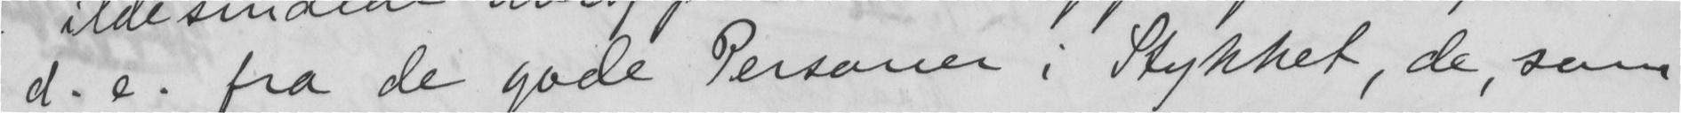- d. e. fra de gode Personer i Stykket, de, som
